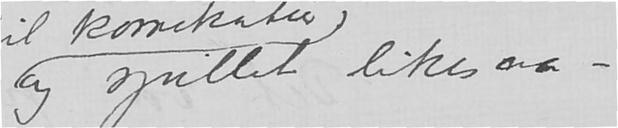og spillet likesaa –
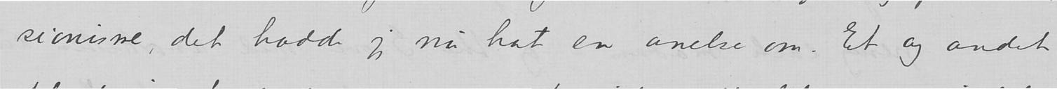ssionisme, det hadde jeg nu hat en anelse om. Et og andet
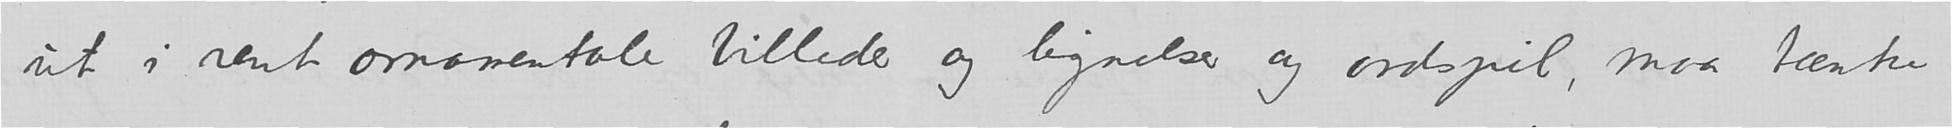ut i rent ornamentale billeder og lignelser og ordspil, maa tænke
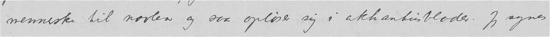menneske til navlen og saa opløser sig i akhanbusblader. Jeg synes
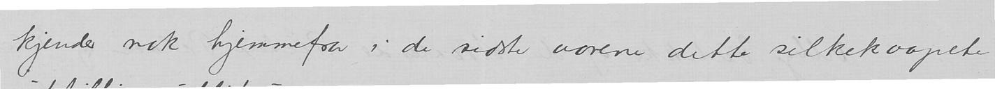kjender nok hjemmefra i det sidste aarene dette silkekaapete
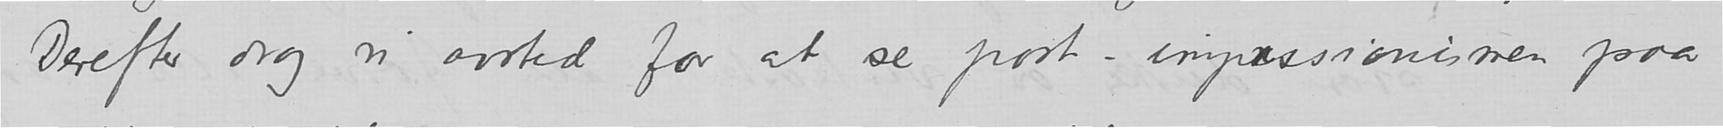Derefter drog vi avsted for at se post-impressionismen paa
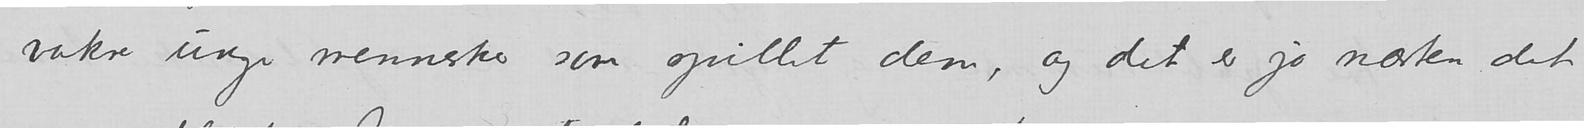vakre unge mennesker som spillet dem, og det er jo næsten det
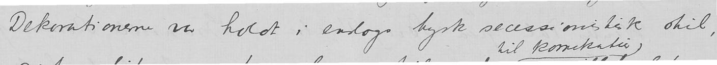Dekorationerne var holdt i enslags kysk secessionistiske stil,
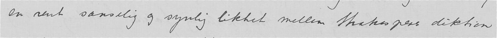en rent sanselig og synlig likhet mellem Shakespers diktion
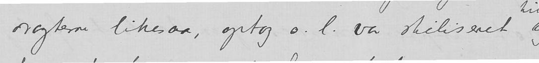dragterne likesaa, optog o.l. var stiliseret
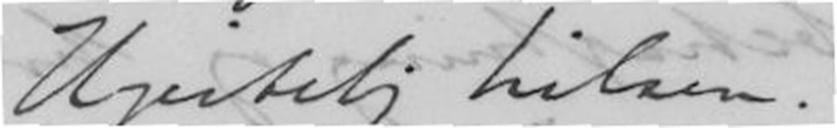Hjertelig hilsen
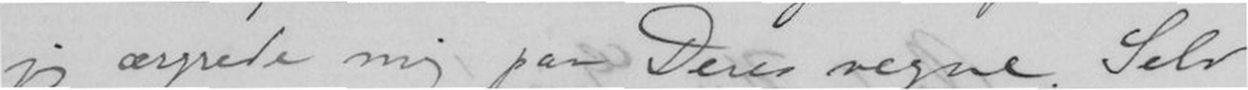jeg ærgrede, mig paa Deres vegne. Selv
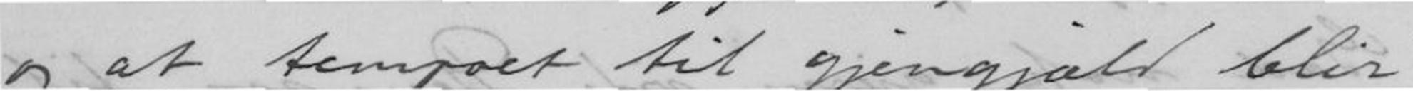og at tempoet til gjengjæld blir
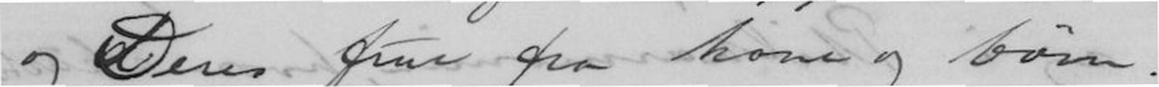og Deres frue fra kone og børn.
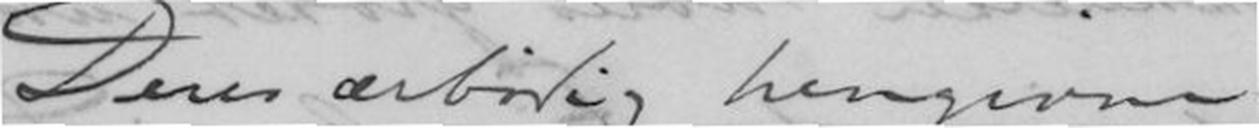Deres ærbødig hengivne
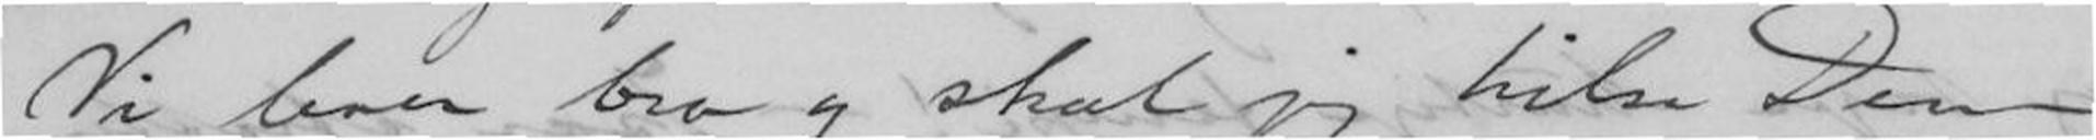Vi lever bra og skal jeg hilse Dem
